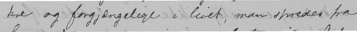kre og forgjængelige i livet, man spredes fra
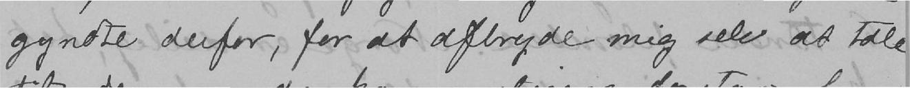gyndte derfor, for at afbryde mig selv at tale
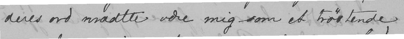deres ord maatte være mig som et trøstende
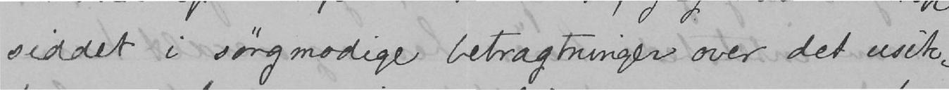siddet i sørgmodige betragtninger over det usik-
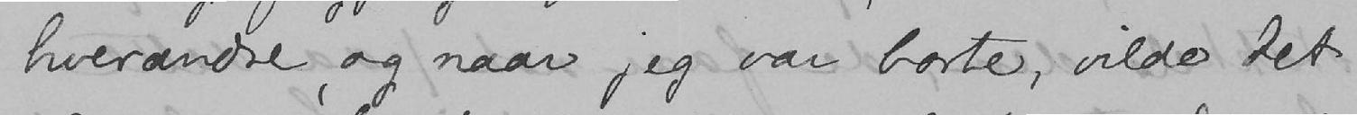hverandre, og naar jeg var borte, vilde det
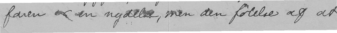faren er en nydelse, men den følelse af at
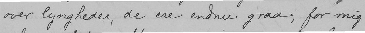over lyngheder, de ere endnu graa, for mig
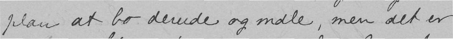plan at bo derude og male, men det er
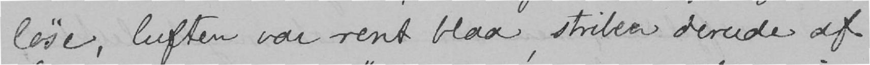løse, luften var rent blaa, striben derude af
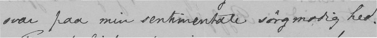svar paa min sentimentale sørgmodighed.
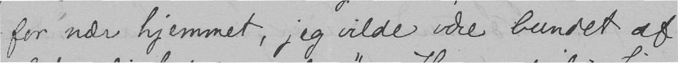for nær hjemmet, jeg vilde være bundet af
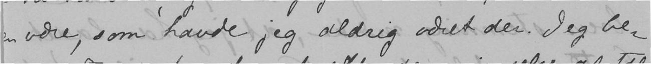være, som havde jeg aldrig været der. Jeg be
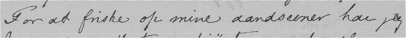For at friske op mine aandsevner har jeg
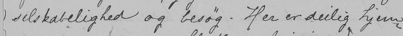selskabelighed og besøg. Her er deilig hjem-
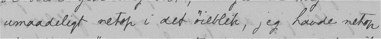umaadeligt netop i det øieblik, jeg havde netop
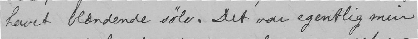havet blændende sølv. Det var egentlig min
